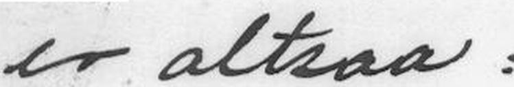er altsaa:
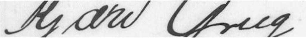Kjære Grieg
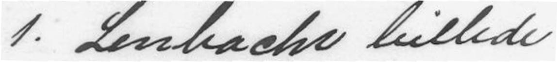1. Lenbachs billede
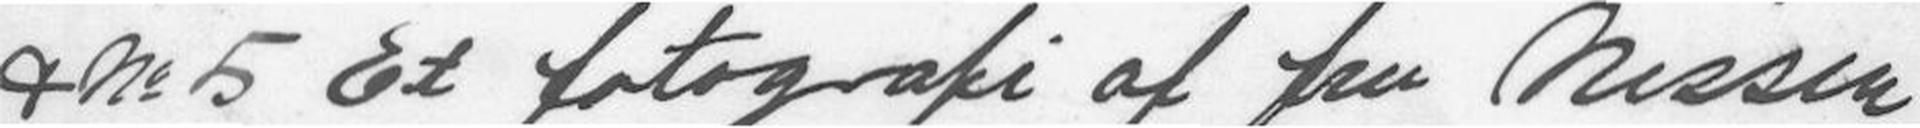& No 5 Et fotografi af fru Nissen
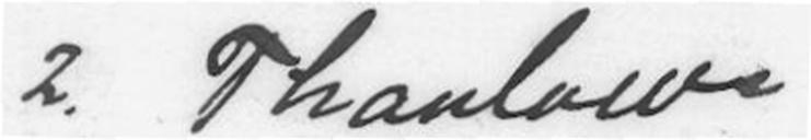2. Thaulows
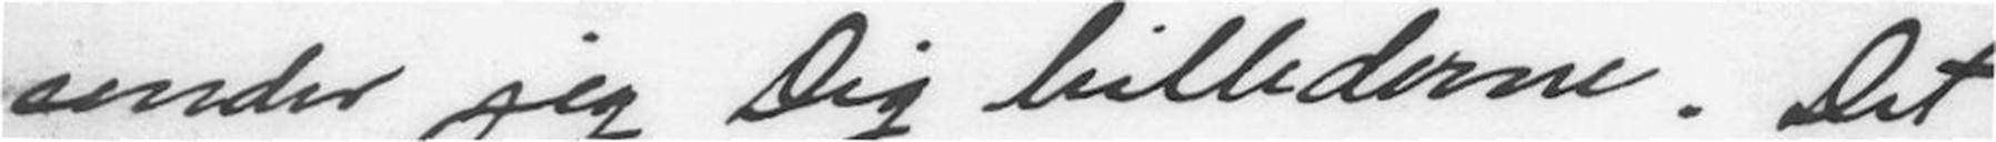sender jeg Dig billederne. Det
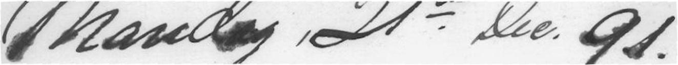Mandag, 21de Dec. 91.
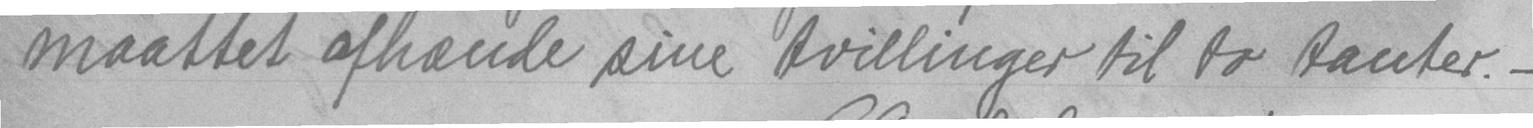maattet afhænde sine tvillinger til to tanter. -
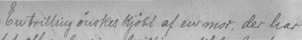En trilling ønskes kjøbt af en mor, der har
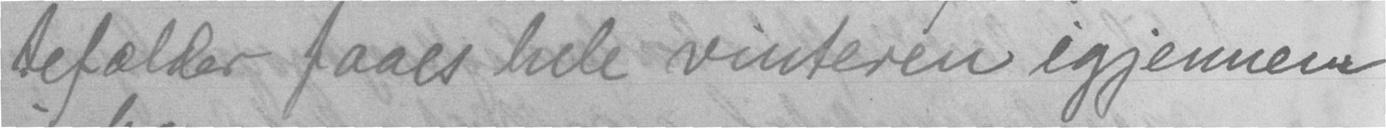tefælder faaes hele vinteren igjennem
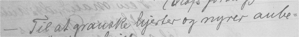- Til at granske hjerter og nyrer anbe-
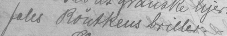fales Røntkens briller. -
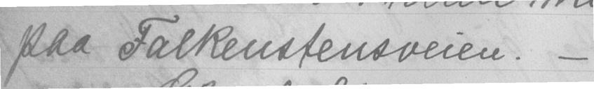paa Falkenstensveien.-
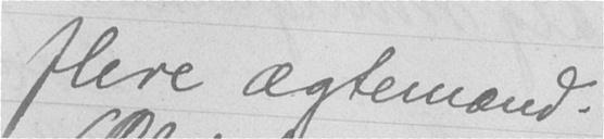flere ægtemænd.
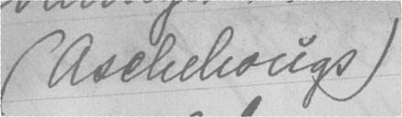(Aschehougs)
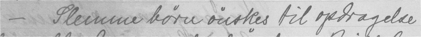- Slemme børn ønskes til opdragelse
